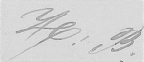H! B.
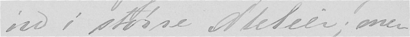ind i større Atelier; men
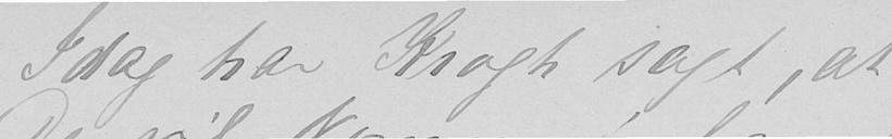Idag har Krogh sagt, at
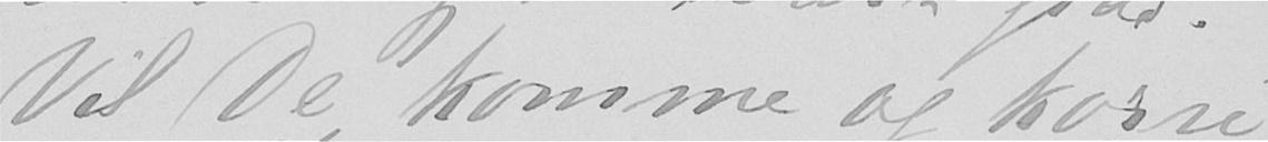Vil De komme og korri-
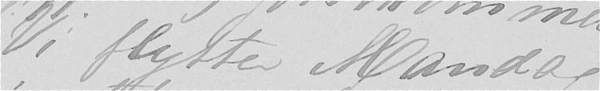Vi flytter Mandag
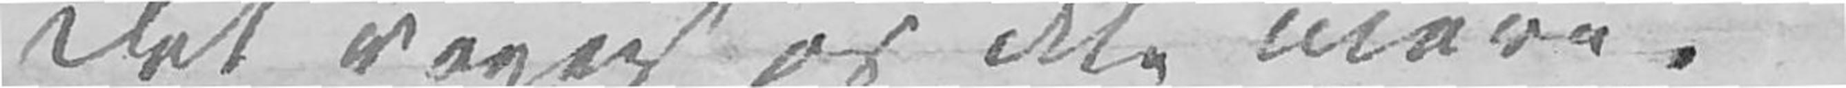Det rager os ikke mere.
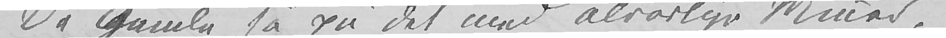De Gamle så på det med alvorlige Miner,
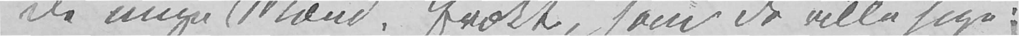de unge Mænd, frækt, som de ville sige:
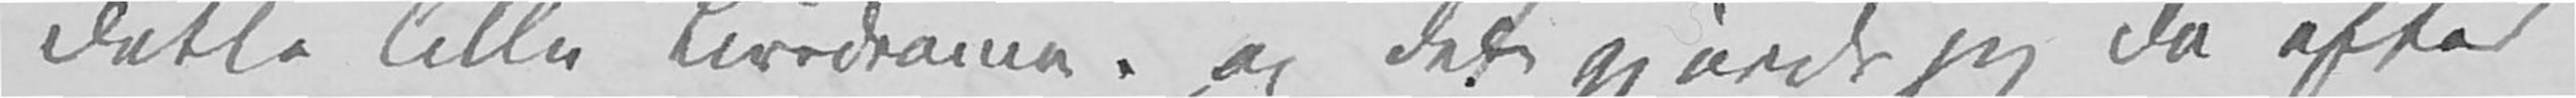dette lille Livsdrama, og det gjorde jeg da efter
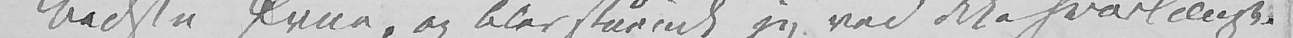bedste Evne, og blev stående jeg ved ikke hvorlænge.
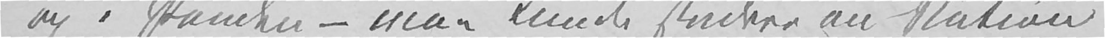op i Panden – man kunde studere en Nation
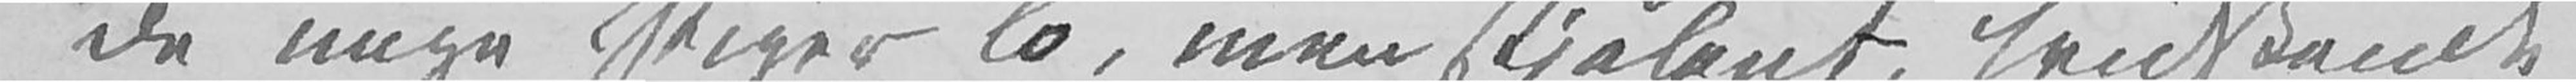de unge Piger lo, men stjålent, hvidskende
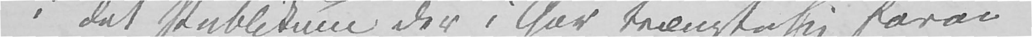i det Publikum der i Går trængte sig foran
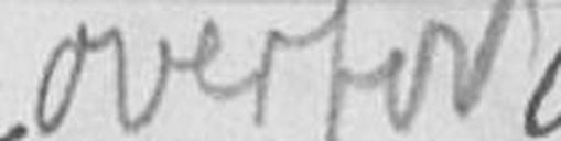overfor
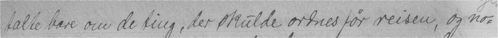talte bare om de ting, der skulde ordnes før reisen, og no-
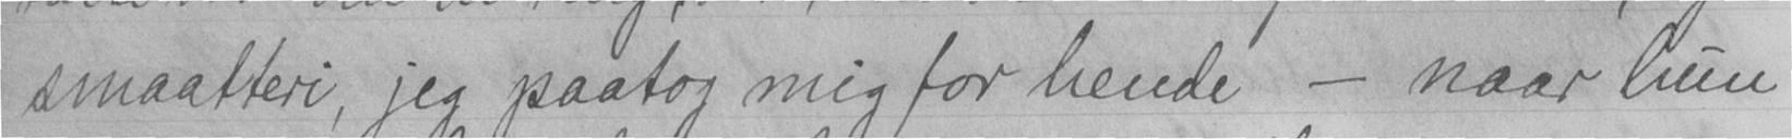smaatteri, jeg paatog mig for hende - naar hun
